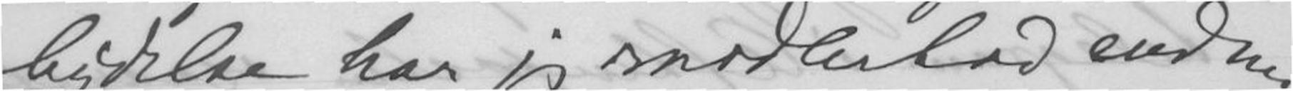bydelse har jeg midlertid endnu
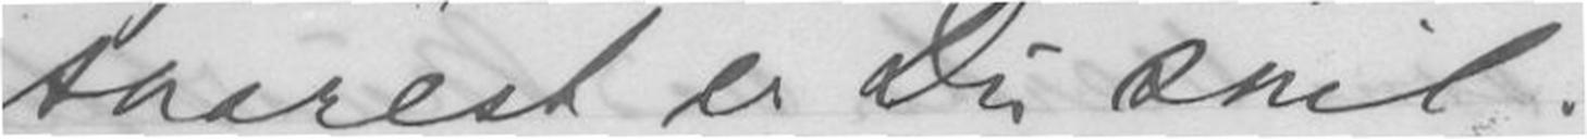snarest er Du snil.
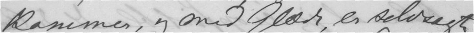kommer, og med Glæde, er selvsagt.
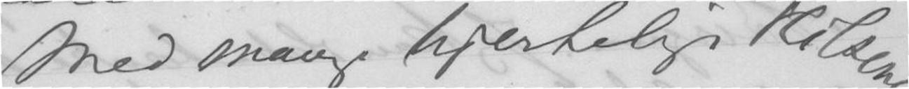Med mange hjertelige Hilsener
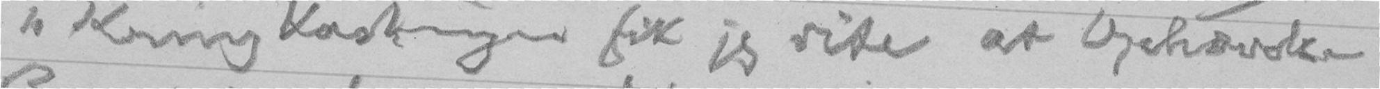"Kringkastingen fik jeg vite at Ophævelsen
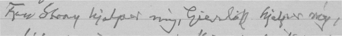Fru Stray hjelper mig, Gierløff hjelper mig,
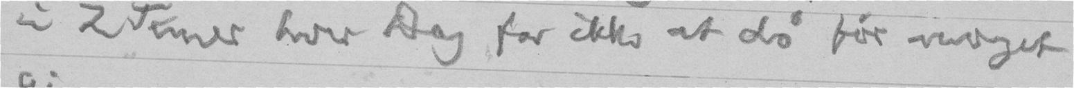i 2 Timer hver Dag for ikke at dø før noget
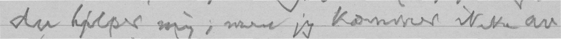du hjelper mig, men jeg kommer ikke av
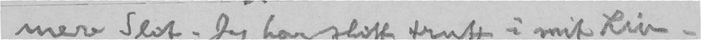mere Slit. Jeg har slitt trutt i mit Liv.
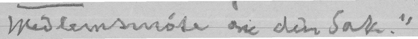Medlemsmøte om den Sak."
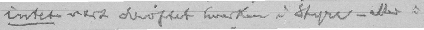intet vært drøftet hverken i Styre - eller i
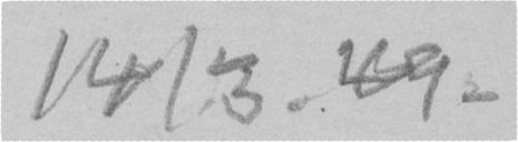14/3. 49.
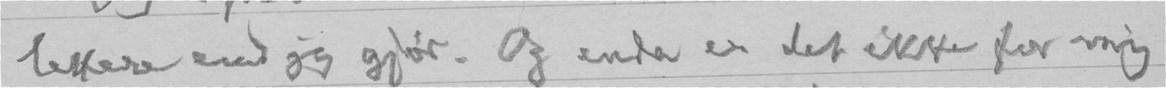lettere end jeg gjør. Og enda er det ikke for mig
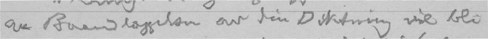av Baandlæggelsen av din Diktning vil bli
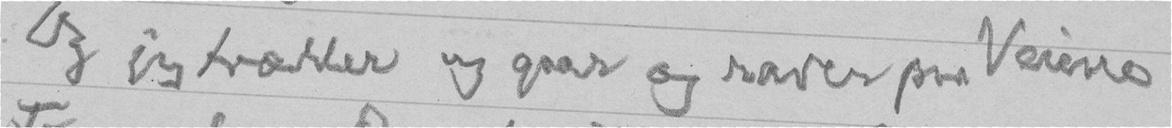Og jeg trætter og gaar og raver paa Veiene
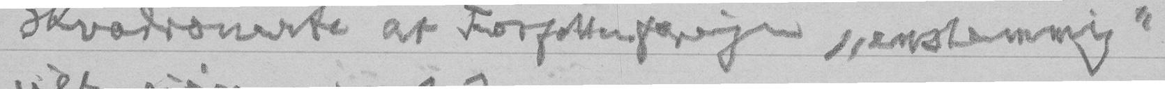skvadronerte at Forfatterforeningen "enstemmig"
